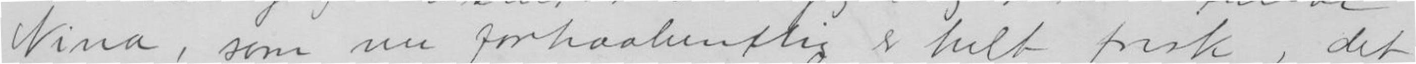Nina, som nu forhaabentlig er helt frisk, det
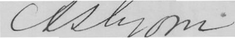Asbjørn
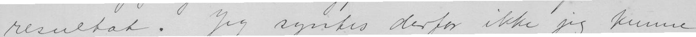resultat. Jeg syntes derfor ikke jeg kunne
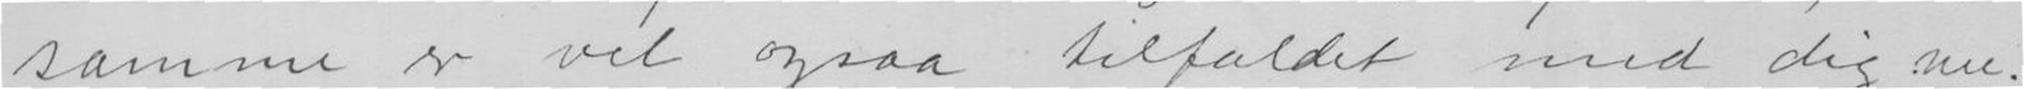samme er vel ogsaa tilfældet med dig nu.
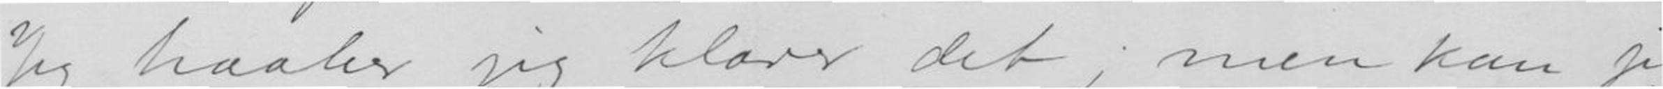Jeg haaber jeg klarer det; men kan jeg
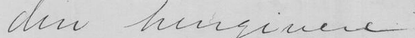din hengivne
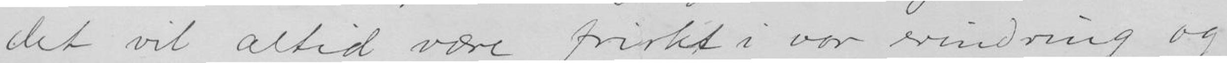det vil altid være friskt i vor erindring og
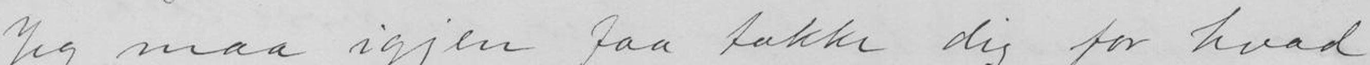Jeg maa igjen faa takke dig for hvad
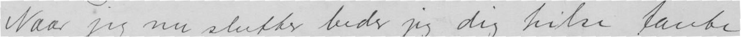Naar jeg nu slutter beder jeg dig hilse tante
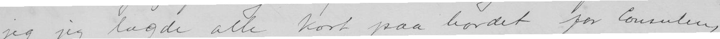jeg jeg lagde alle kort paa bordet for Consulen,
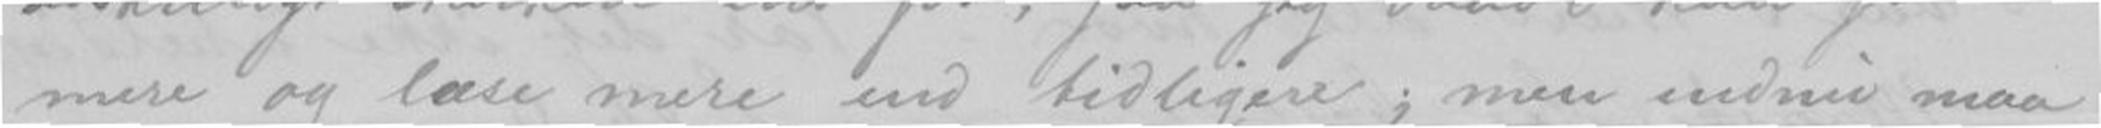mere og læse mere end tidligere; men endnu maa
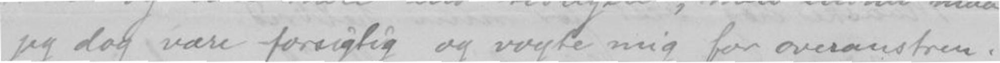jeg dog være forsigtig og vogte mig for overanstren-
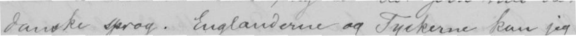danske sprog. Englænderne og Tyskerne kan jeg
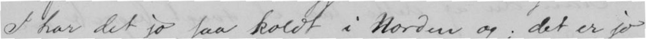I har det jo saa koldt i Norden og, der er jo
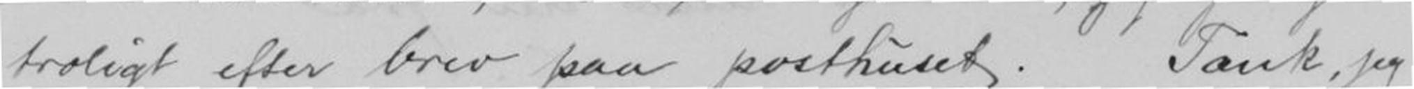troligt efter brev paa posthuset. Tænk, jeg
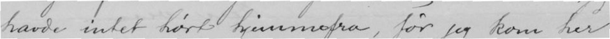havde intet hørt hjemmefra, før jeg kom her
